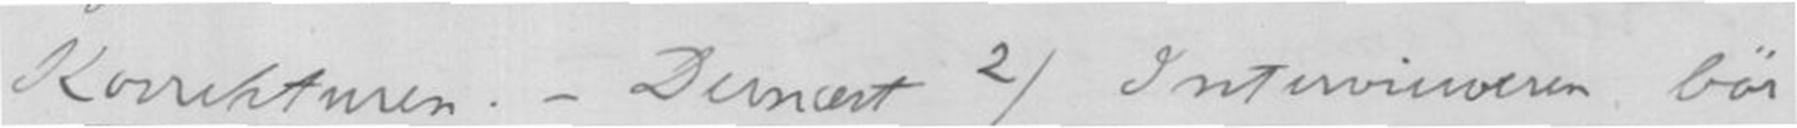Korekturen. - Dernæst 2) Intervieweren bør
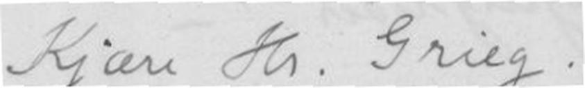Kjære Hr. Grieg.
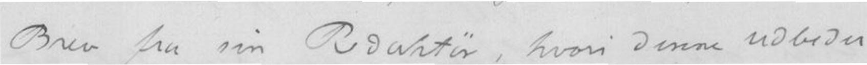Brev fra sin Redaktør, hvori denne udbeder
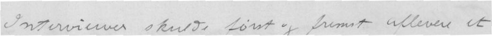Interviewer skulde først og fremst aflevere et
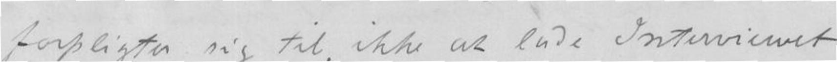forpligter sig til, ikke at lade Interviewet
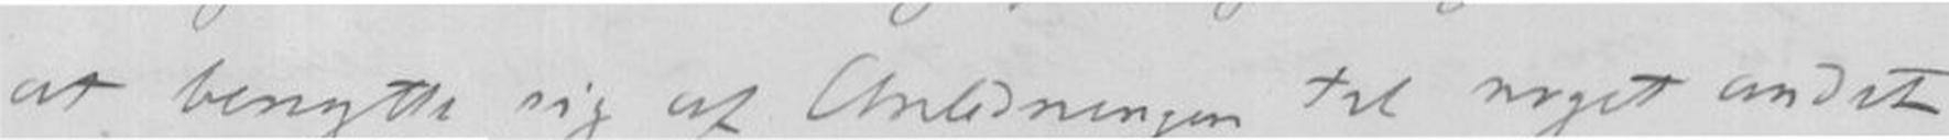at benytte sig af Anledningen til noget andet
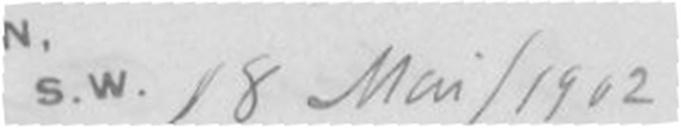S.W. 18 Mai/ 1902
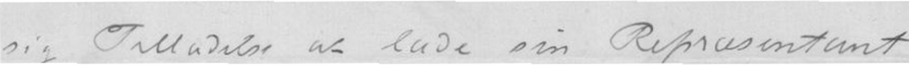sig Tilladelse at lade sin Repræsentant
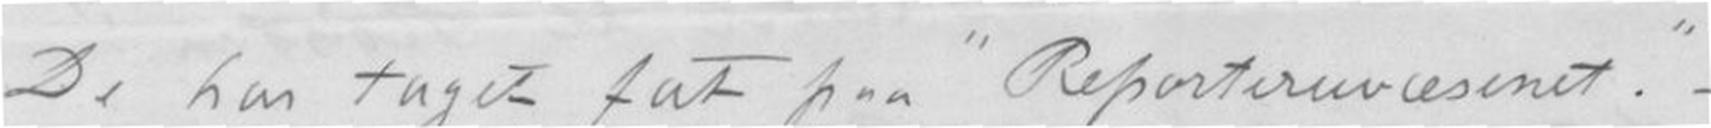De har taget fat paa "Reporteruvæsenet." -
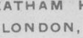LONDON,
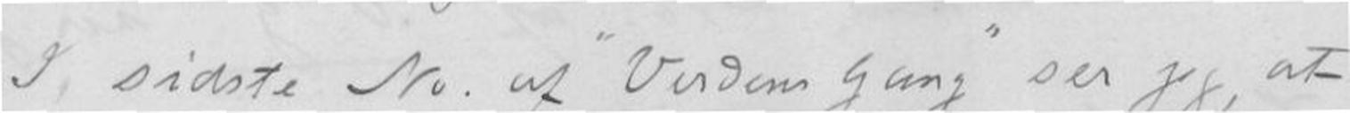I sidste No. af "Verdens Gang" ser jeg, at
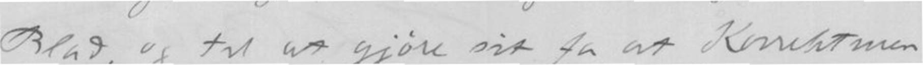Blad, og til at gjøre sit for, at korrekturen
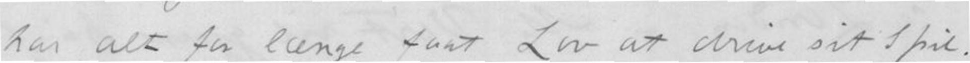har alt for længe faat Lov at drive sit Spil.
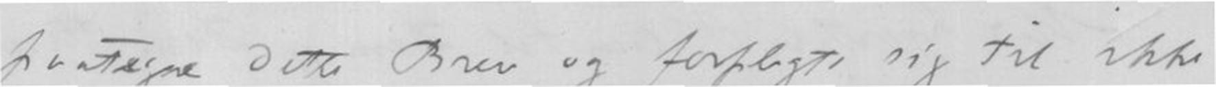paategne dette Brev og forpligte sig til ikke
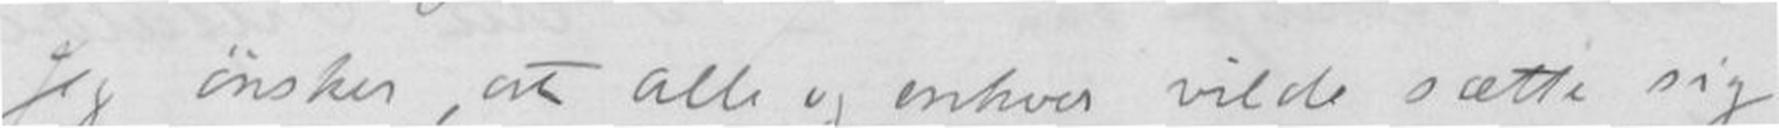Jeg ønsker, at alle og enhver vilde sætte sig
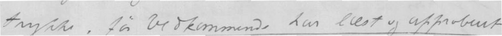trykke. før Vedkommende har læst og appsoberet
